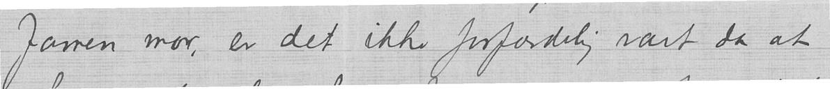Jamen mor, er det ikke forfærdelig rart da at
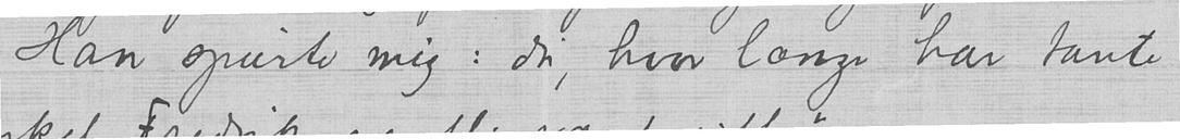Han spurte mig: du, hvor længe har tante
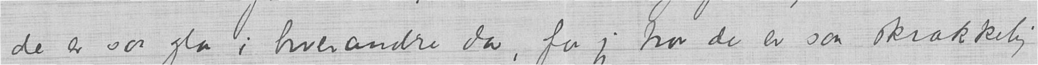de er saa gla i hverandre da, for jeg tror de er saa skrækkelig
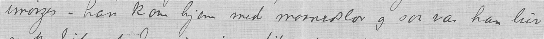imorges – han kom hjem med maanedslov og saa var han lur
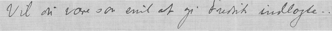Vil du være saa snil at gi Fredrik indlagte -.
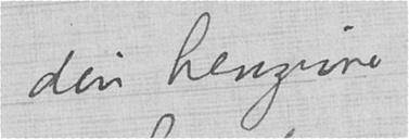din hengivne
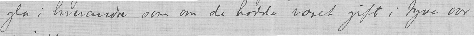gla i hverandre som om de hadde været gift i tyve aar
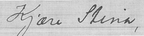Kjære Stina,
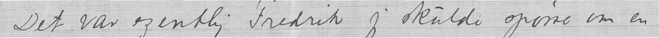Det var egentlig Fredrik jeg skulde spørre om en
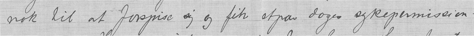nok til at forspise sig og fik etpar dages sykepermission.
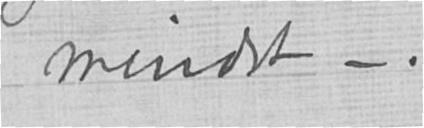mindst –.
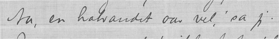Aa, en halvandet aar vel, sa jeg.
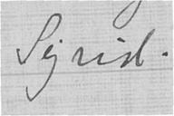Sigrid.
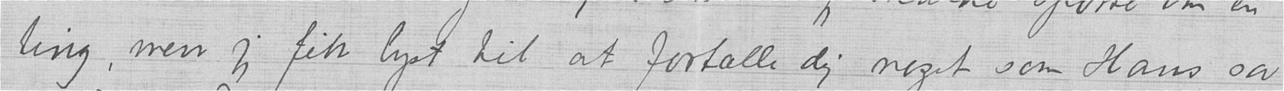ting, men jeg fik lyst til at fortælle dig noget som Hans sa
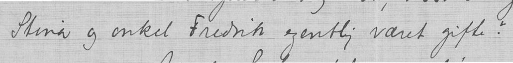Stina og onkel Fredrik egentlig været gifte?
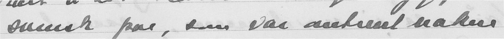svensk par, som var omtrent nakne
